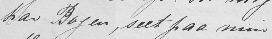har Bogen, seet paa min
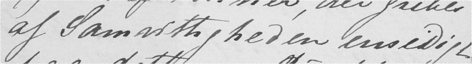af Samvittigheden ensidigt
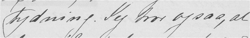tydning. Jeg tror ogsaa, at
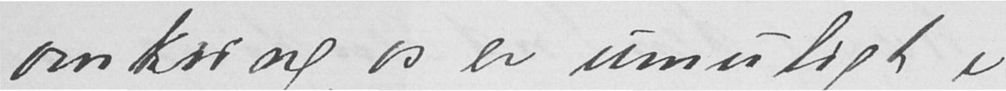omkring os er umuligt i
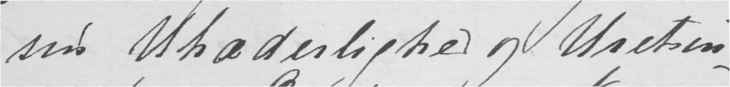sin Uhæderlighed og Uretsin-
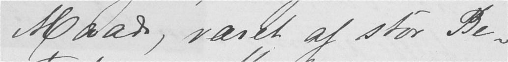Maade, været af stor Be-
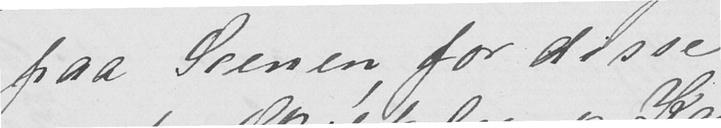paa Scenen, for disse
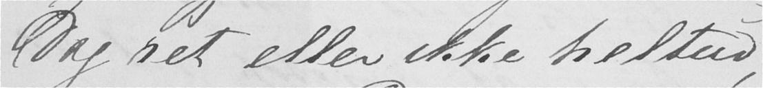Dig ret eller ikke heltud,
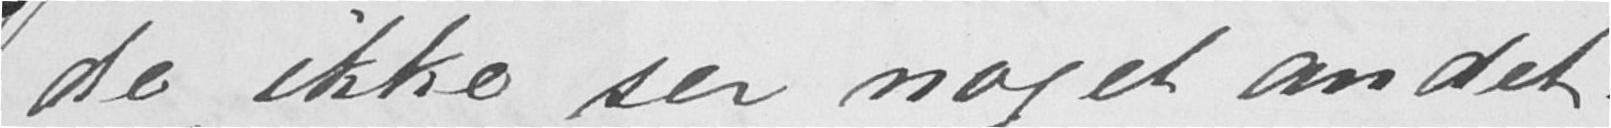de ikke ser noget andet.
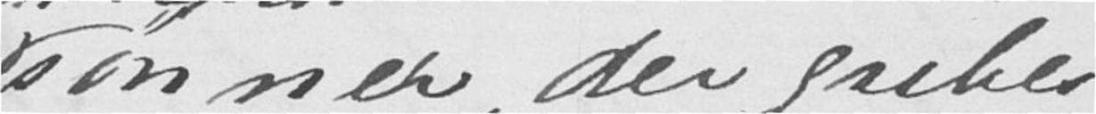sønner, der gribes
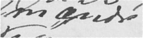mænds
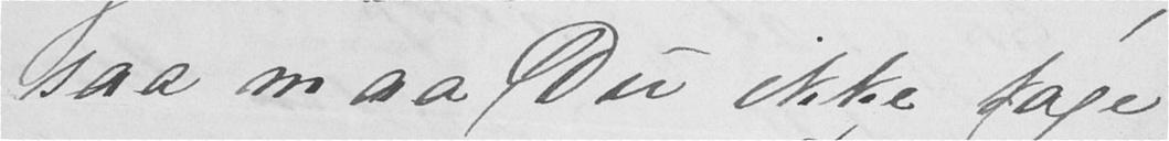saa maa Du ikke tage
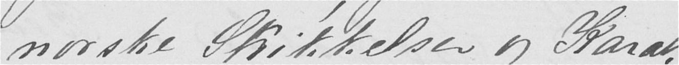norske Skikkelser og Karak-
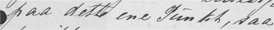paa dette ene Punkt, saa
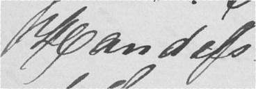Handels
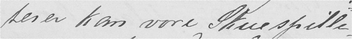terer kan vore Skuespille-
